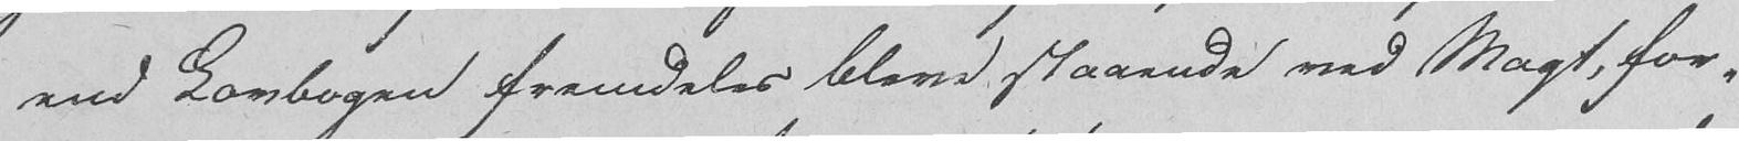end Lovbogen fremdeles bleve staaende ved Magt, for-
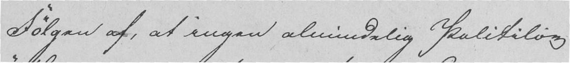Følgen af, at ingen almindelig Politilov
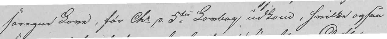særegne Love, før Chr d. 5tes Lovbog udkom, hvilke ogsaa
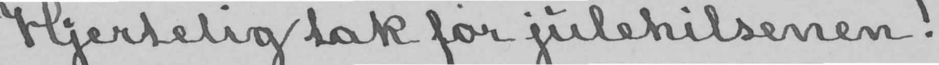Hjertelig tak for julehilsenen!
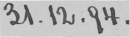31.12.94.
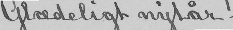Glædeligt nytår!
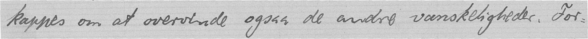kappes om at overvinde ogsaa de andre vanskeligheder. For-
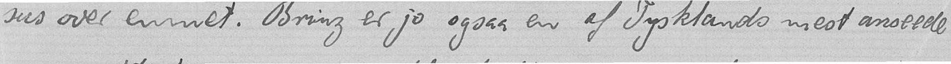sus over emnet. Brinz er jo ogsaa en af Tysklands mest anseede
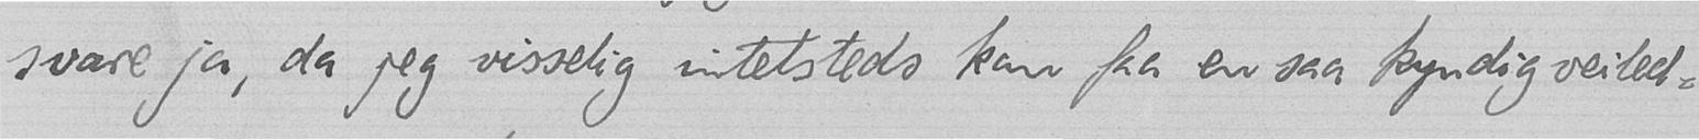svare ja, da jeg visselig intetsteds kan faa en saa kyndig veiled-
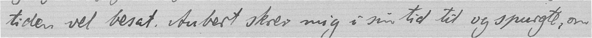tiden vel besat. Aubert skrev mig i sin tid til og spurgte, om
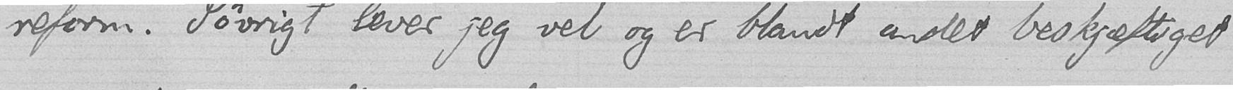reform. Iøvrigt lever jeg vel og er blandt andet beskjæftiget
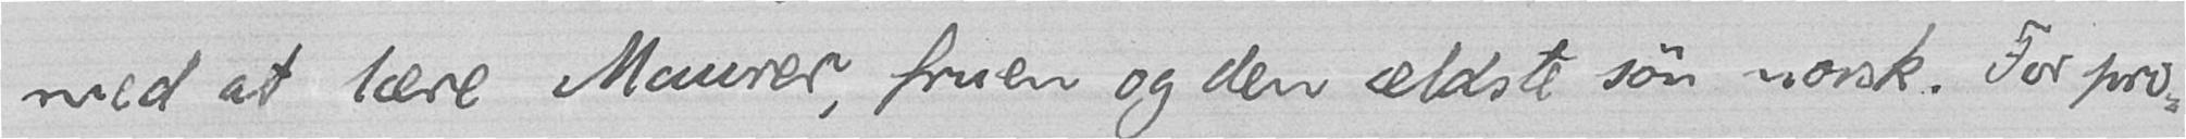med at lære Maurer, fruen og den ældste søn norsk. For pro-
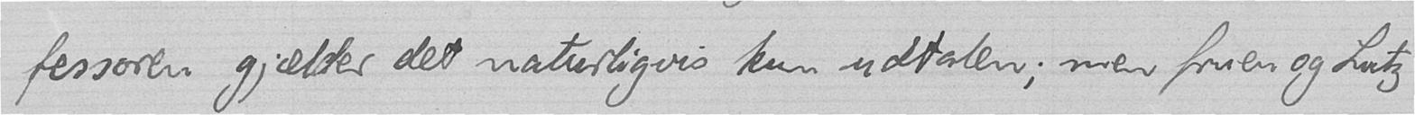fessoren gjælder det naturligvis kun udtalen; men fruen og Lutz
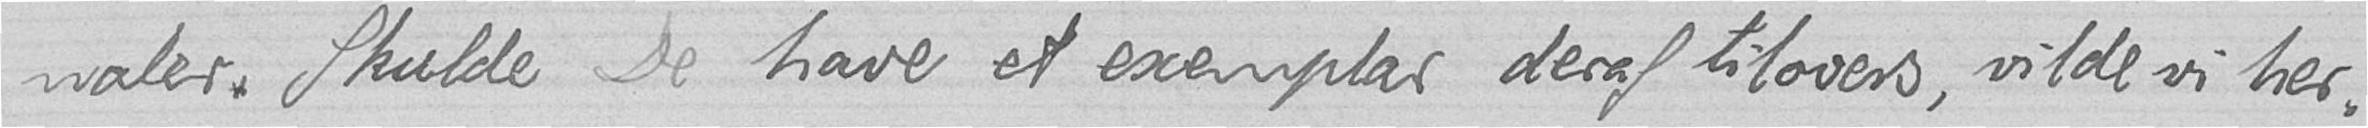naler. Skulde De have et exemplar deraf tilovers, vilde vi her-
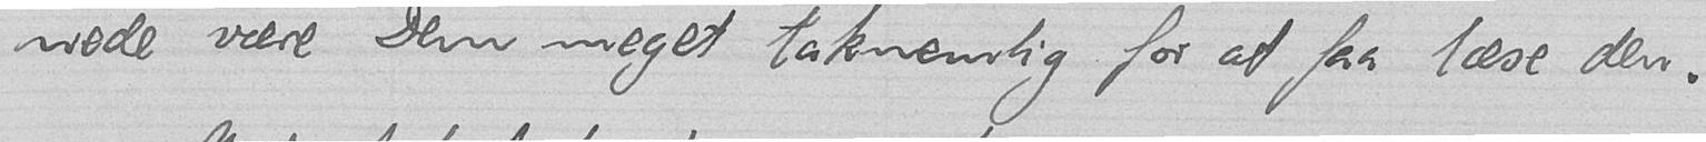nede være Dem meget taknemlig for at faa læse den.
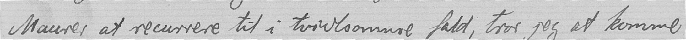Maurer at recurrere til i tvivlsomme fald, tror jeg at komme
